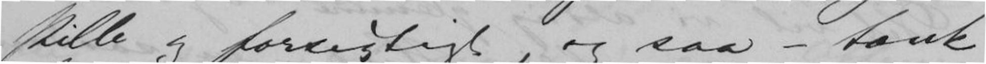stille og forsigtigt, og saa - tænk
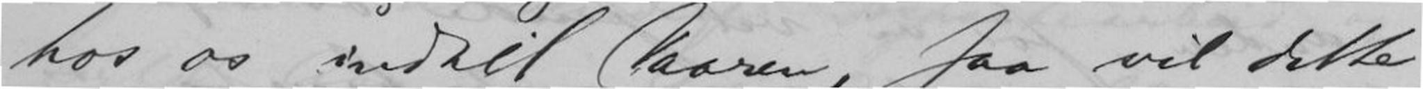hos os indtil Vaaren, saa vil dette
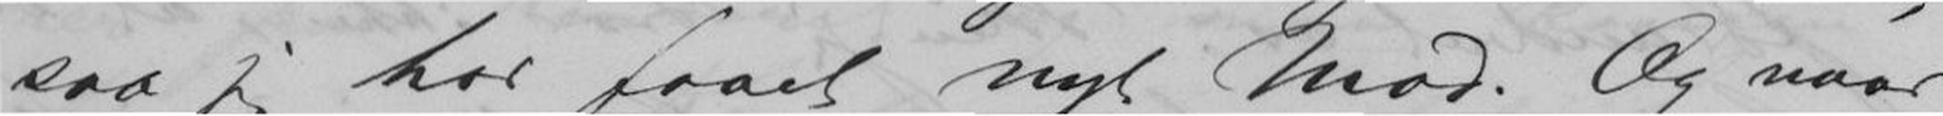saa jeg har faaet nyt Mod. Og naar
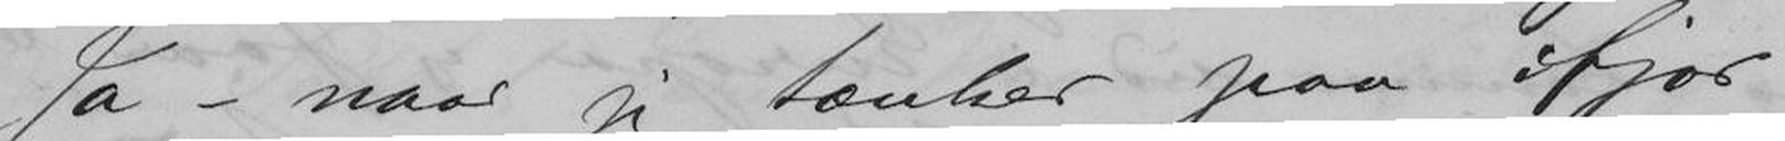Ja, naar jeg tænker paa ifjor -
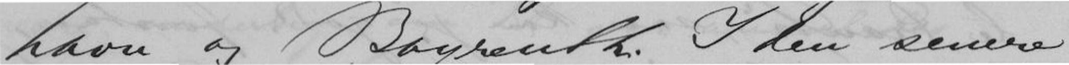havn og Bayreuth. I den senere
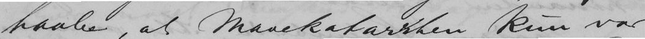haabe, at Mavekatarrhen kun var
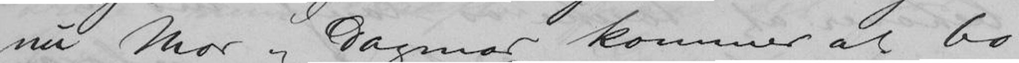nu Mor og Dagmar kommer at bo
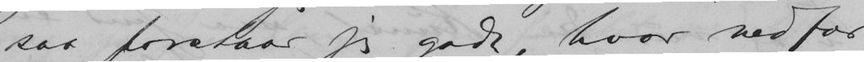saa forstaar jeg godt, hvor nedfor
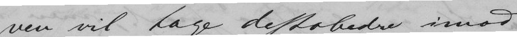ven vil tage destobedre imod
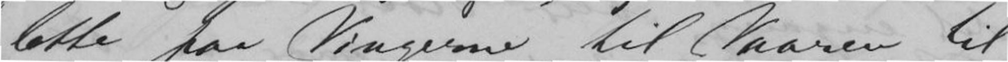lette paa Vingerne til Vaaren til
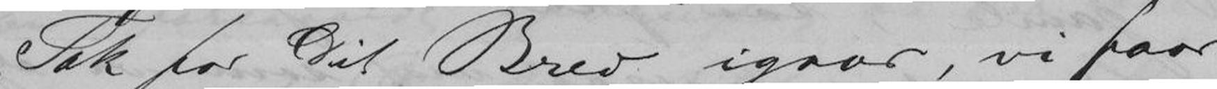Tak for Dit Brev igaar, vi faar
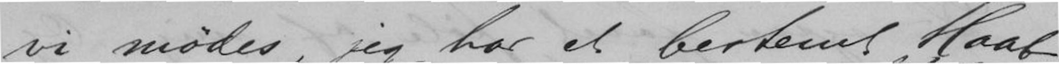vi mødes, jeg har et bestemt Haab
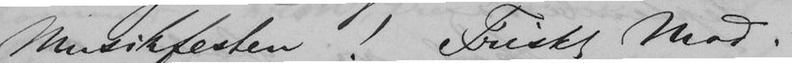Musikfesten! Friskt Mod!
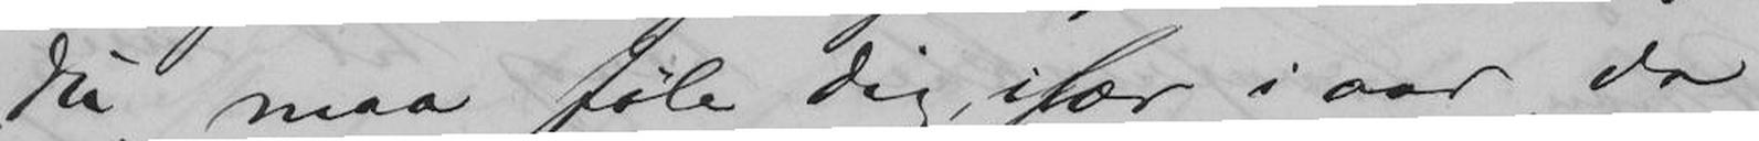Du maa føle dig, især i aar da
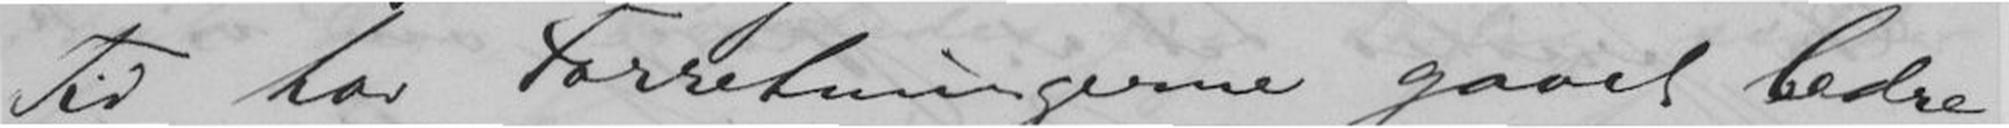Tid har Forretningerne gaaet bedre,
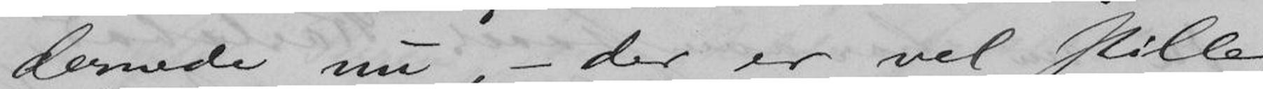dernede nu, - der er vel stille.
